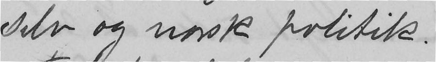selv og norsk politik.
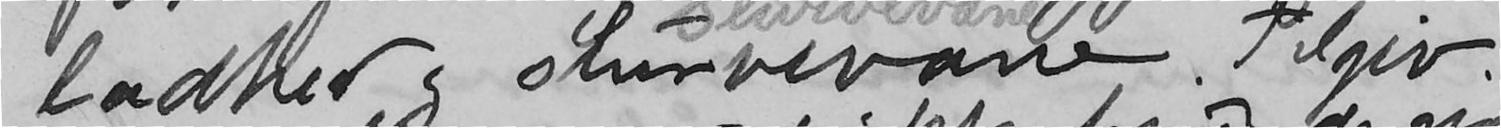ladhed og slurvevane. Tilgiv
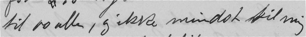til os alle, og ikke mindst til mig
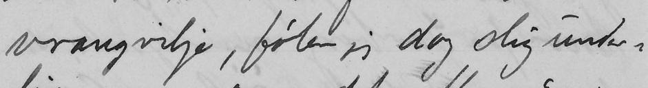vrangvilje, føler jeg dog slig under-
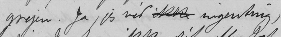grejen. Ja, jeg véd ikke ingenting,
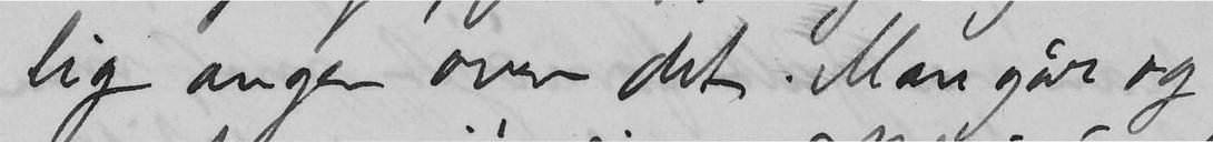lig anger over det. Man går og
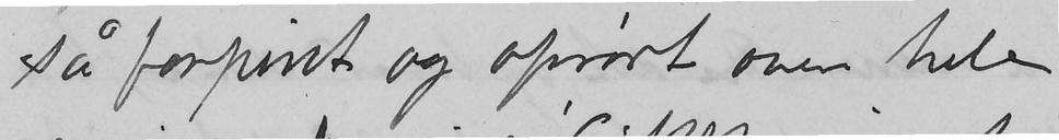så forpint og oprørt over hele
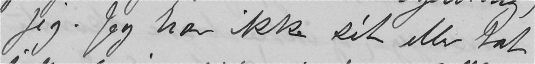jeg. Jeg har ikke sét eller tat
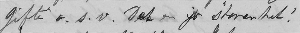gifte" o.s.v. Det er jo storartet!
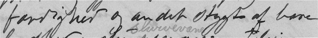færdighed og andet stygt af bare
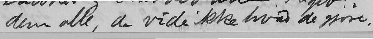dem alle, de vide ikke hvad de gjøre.
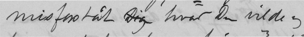misforståt Dig hvad Du vilde og
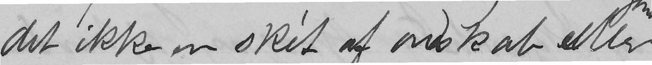det ikke er skét af ondskab eller
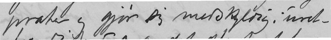prater og gjør sig medskylddig i uret-
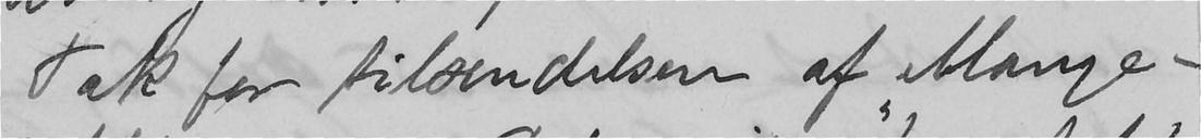Tak for tilsendelsen af "Mange-
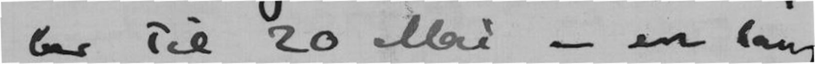ber til 20 Mai - en lang
